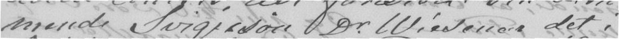mende Svigersøn Dr Wiesener det i
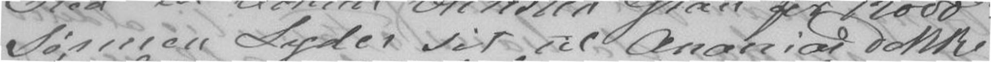Sønnen Lyder sit til Ananias Dokke
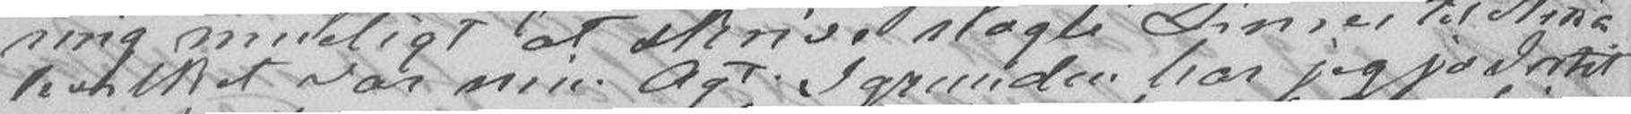hvilket var min Agt. Igrunden har jeg jo Intet
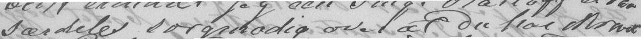særdeles sorgmodig over at Du har skrevet
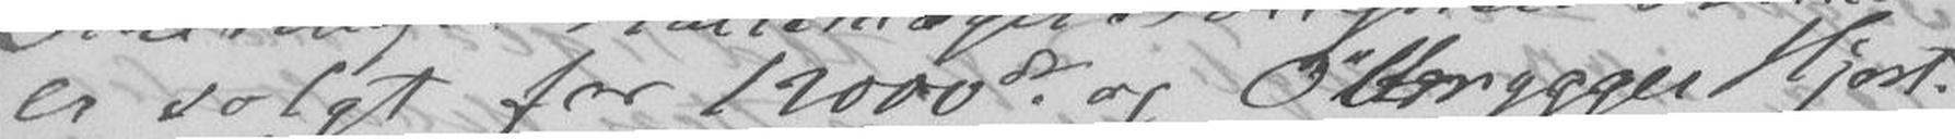er solgt for 12000do og Ølbrygger Hjort-
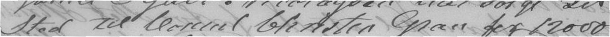Sted til Concul Christen Gran for 12000
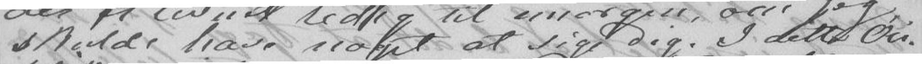skulde have noget at sige Dig. I dette Øie-
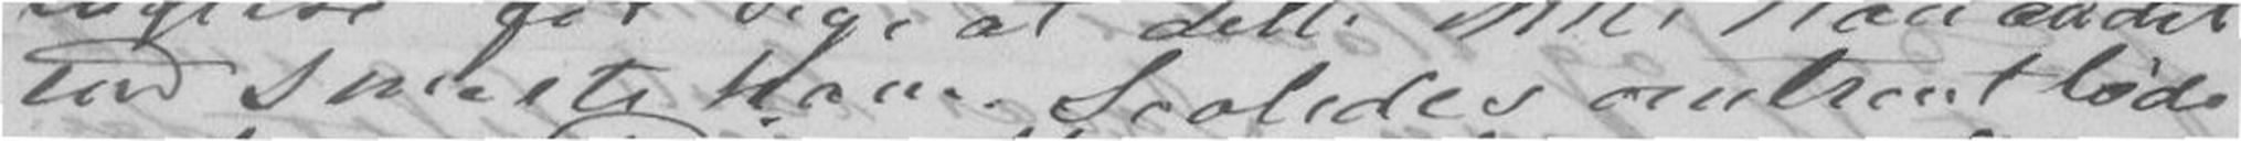end smerte ham. Saaledes omtrent lød
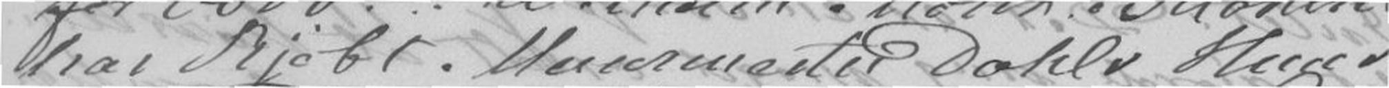har Kjøbt Murermester Dahls Huus
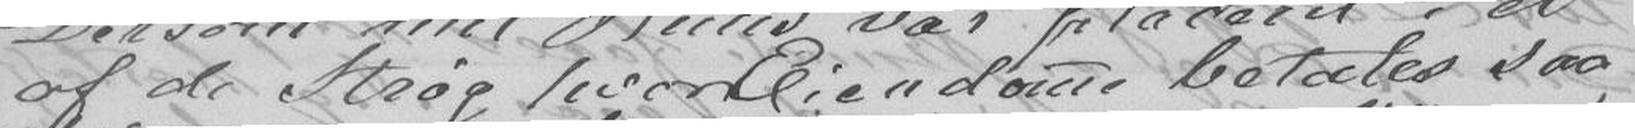af de Strøk hvor Eiendo`me betales saa
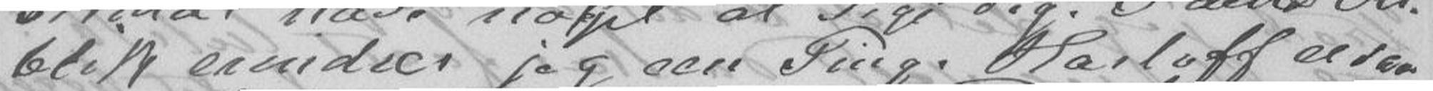blik erindrer jeg een Ting. Harloff er saa
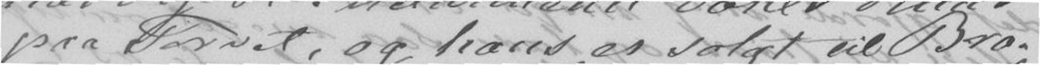paa Torvet, og hans er solgt til Bro-
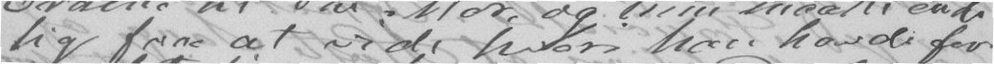lig faae at vide hvori han havde for-
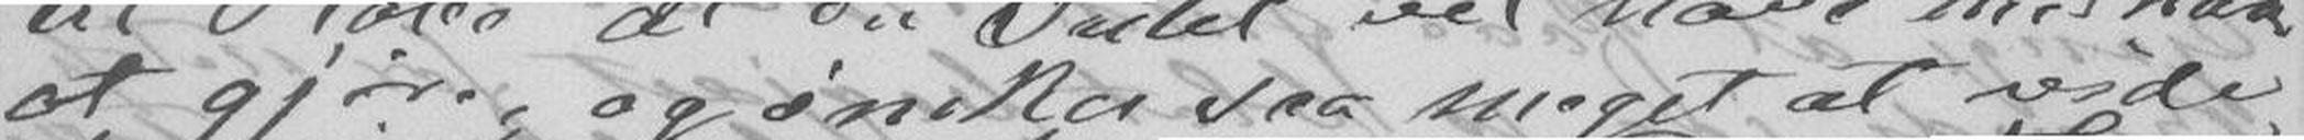at gjøre, og ønsker saa meget at vide
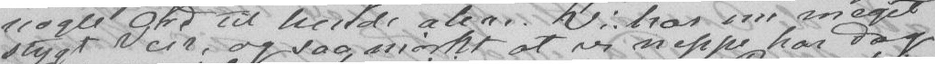stygt Veir, og saa mørkt at vi neppe har Dag.
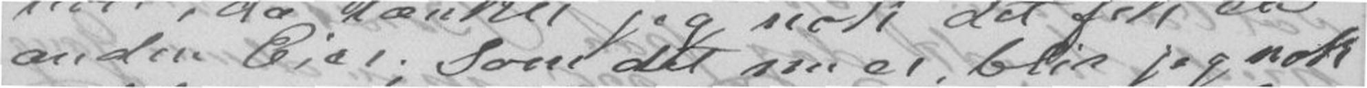anden Eier. Som det nu er blir jeg nok
